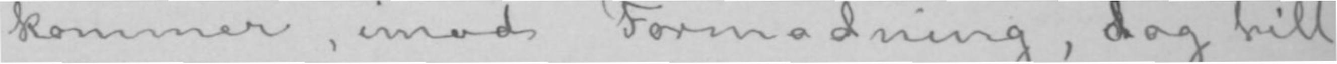kommer, imod Formodning, dog till
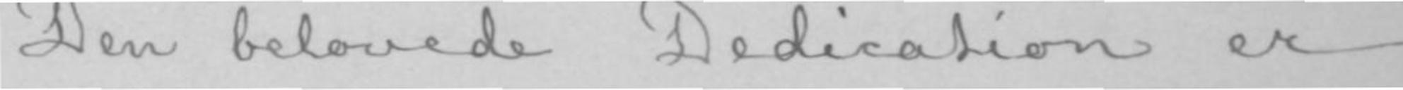Den belovede Dedication er
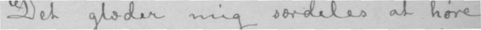Det glæder mig særdeles at høre
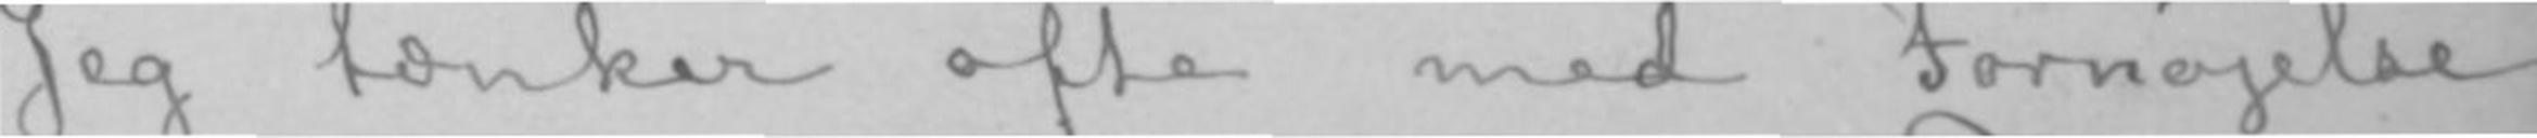Jeg tænker ofte med Fornøjelse
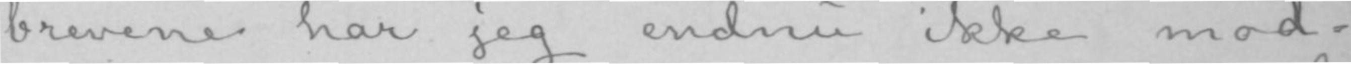brevene har jeg endnu ikke mod-
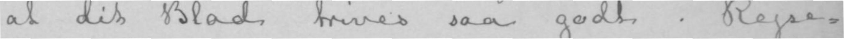at dit Blad trives saa godt. Rejse-
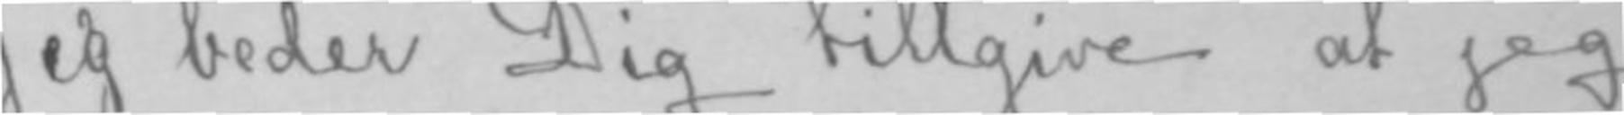Jeg beder Dig tillgive at jeg
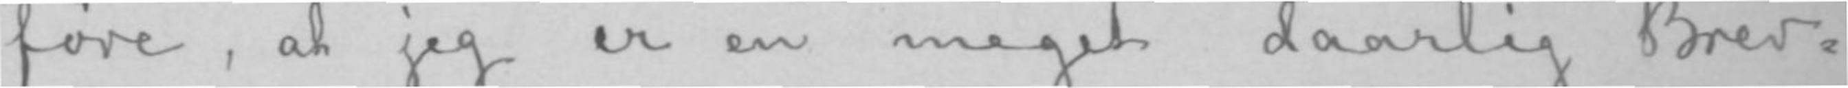føre, at jeg er en meget daarlig Brev-
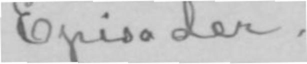Episoder.
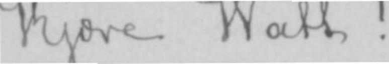Kjære Watt!
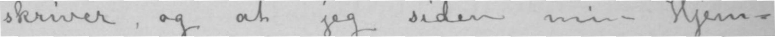skriver, og at jeg siden min Hjem-
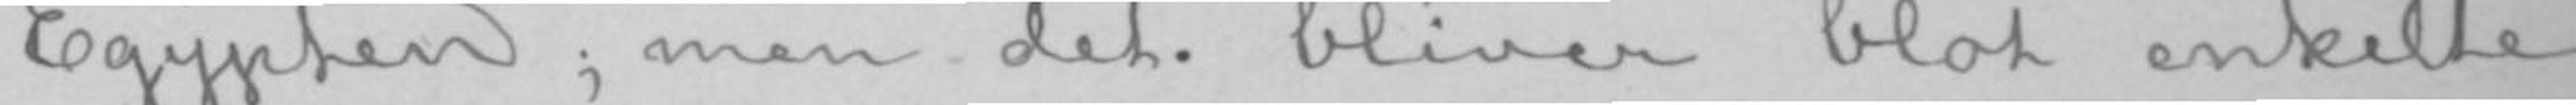Egypten; men det bliver blot enkelte
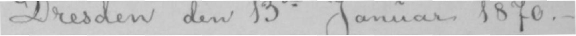Dresden den 13de Januar 1870.-
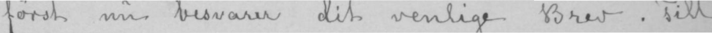først nu besvarer dit venlige Brev. Till
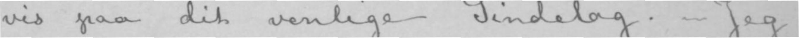vis paa dit venlige Sindelag.- Jeg
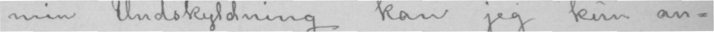min Undskyldning kan jeg kun an-
